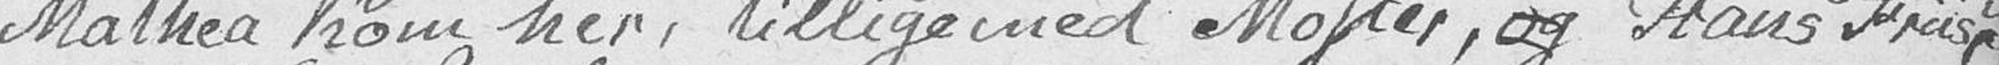Mathea kom her, tilligemed Moster, og Hans Friis,
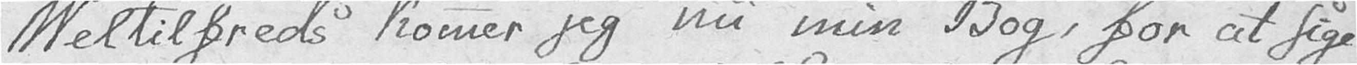Veltilfreds kommer jeg nu min Bog, for at sige
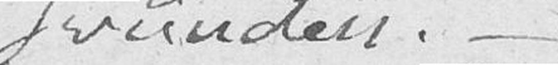svunden. –
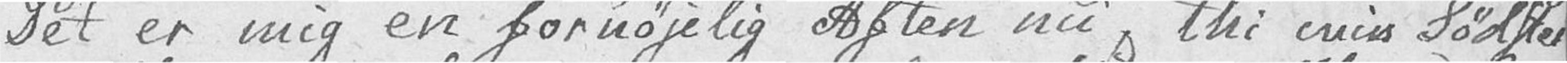Det er mig en fornøjelig Aften nu, thi min Sødster
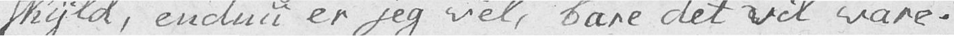skyld, endnu er jeg vel, bare det vil vare.
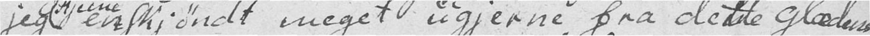jeg Hjemme enskjøndt meget ugjerne fra dette glædens
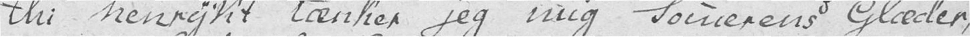thi henrykt tænker jeg mig Sommerens Glæder,
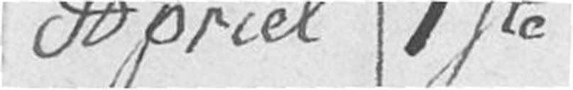Apriel 1ste
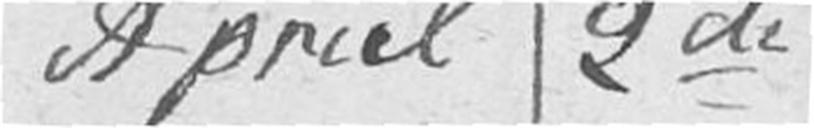Apriil 2de
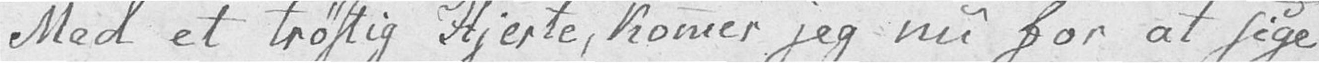Med et trøstig Hjerte, kommer jeg nu for at sige
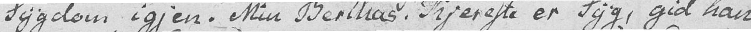Sygdom igjen. Min Berthas! Kjereste er Syg, gid han
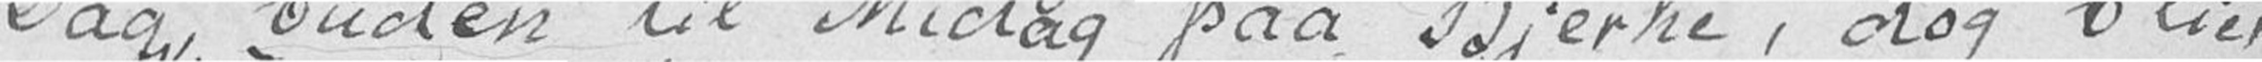Dag buden til Midag paa Bjerke, dog blier
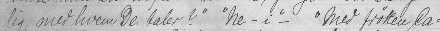lig, med hvem De taler?" "Ne - i" - "Med frøken Ca-
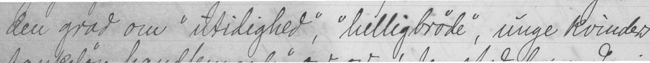den grad om "utidighed", "helligbrøde", unge kvinders
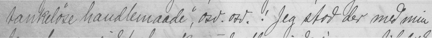tankeløse handlemaade", osv. osv.! Jeg stod der med min
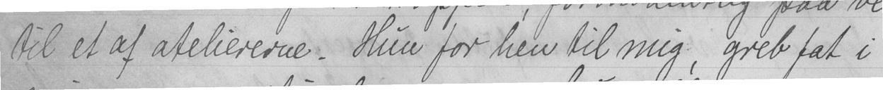til et af ateliererne. Hun for hen til mig, greb fat i
